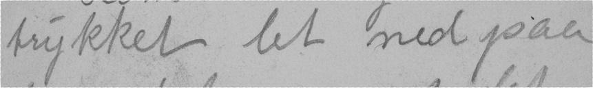trykket let ned paa
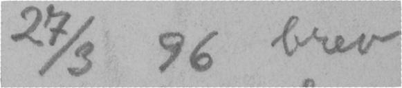27/3 96 brev
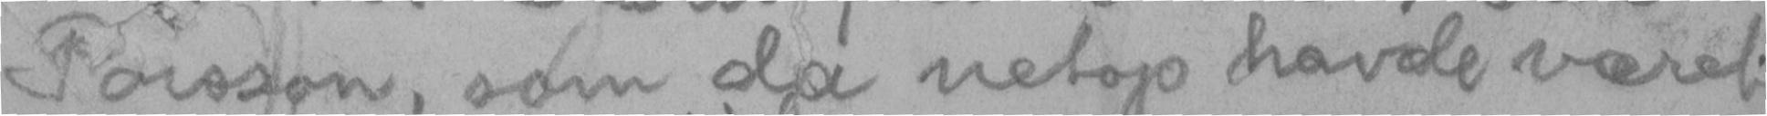Poisson, som da netop havde været
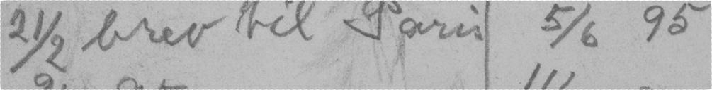2 1/2 brev til Paris 5/6 95
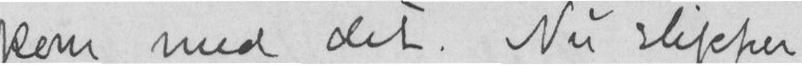kom med det, Nu slipper
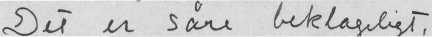Det er såre beklageligt,
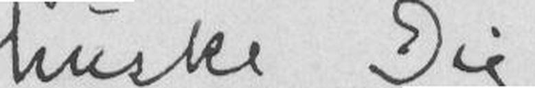huske Dig
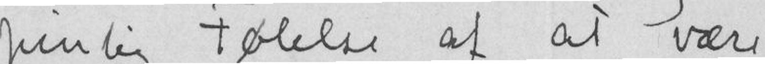pinlig Følelse af at være
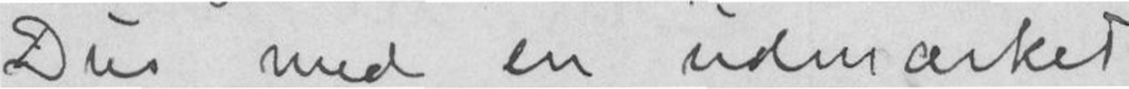Dus med en udmærket
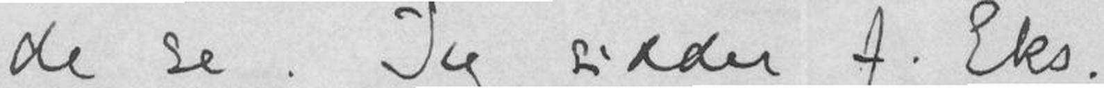de se. Jeg sidder f. Eks.
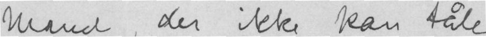Mand, der ikke kan tåle
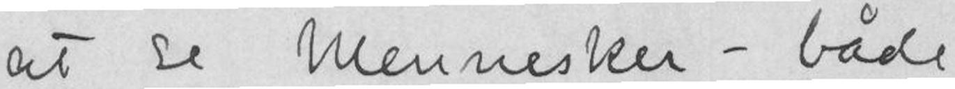at se Mennesker - både
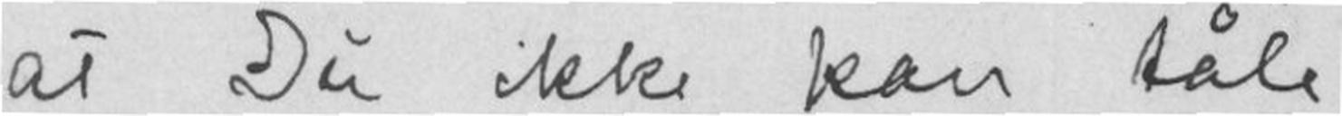at Du ikke kan tåle
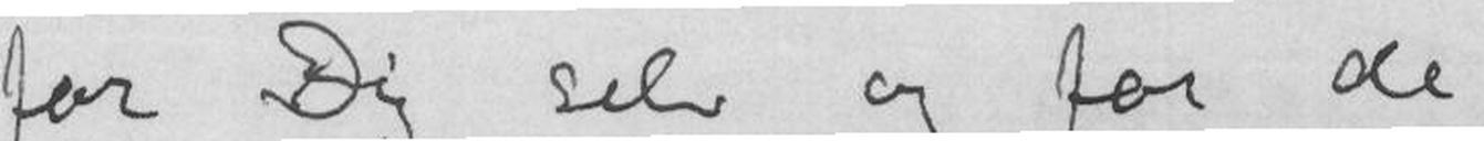for Dig selv og for de
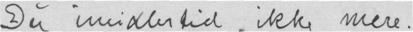Du imidlertid ikke mere.
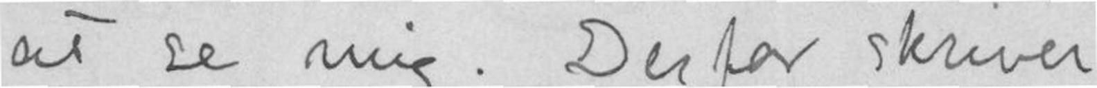at se mig. Derfor skriver
